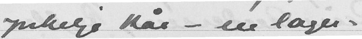opkelige kår - en lager-
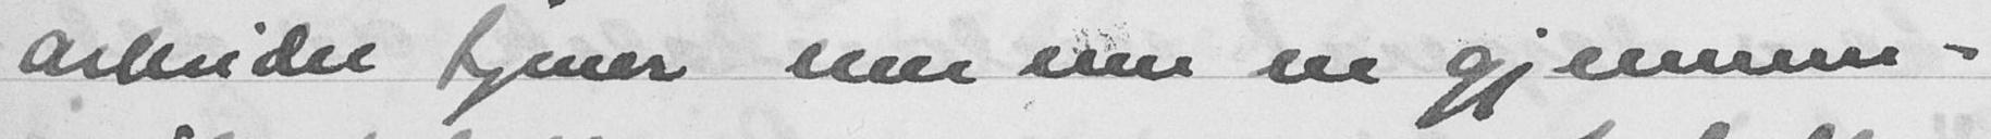arbeider tjener mer enn en gjennem-
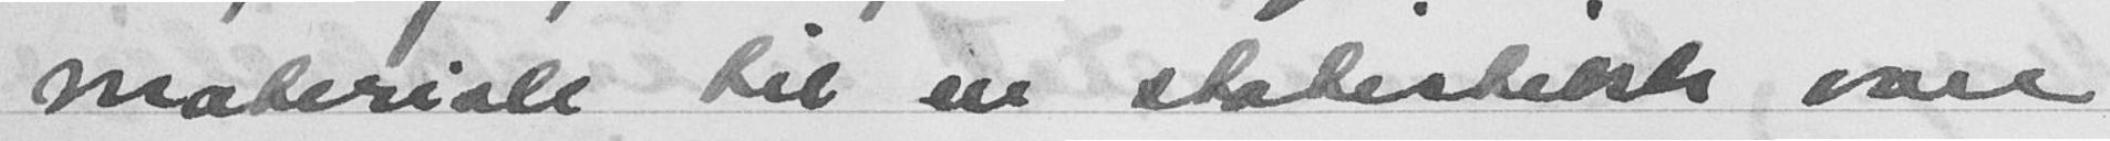materiale til en statistikk over
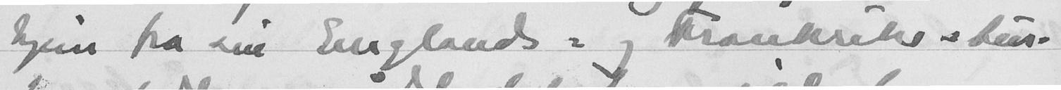hjem fra sin Englands- og Frankrikes-tur.
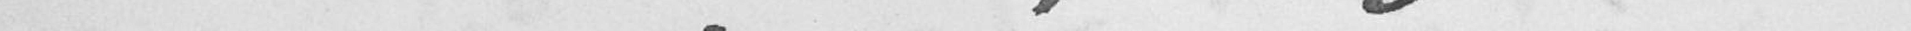arbeider tjener mer enn en gjennem-
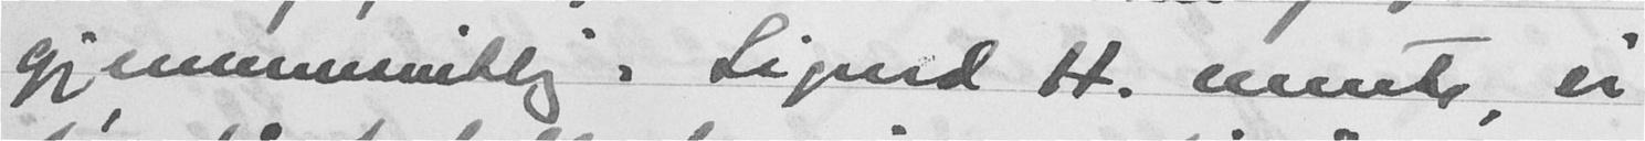gjennomsnitlig, Sigurd H. mente, vi
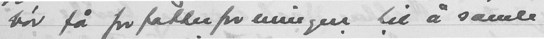bør få forfatterforeningen til å samle
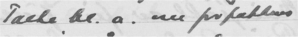Talte bl. a. om forfatternes
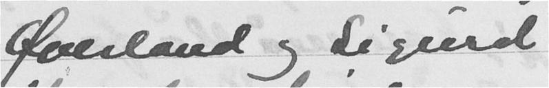Øverland og Sigurd
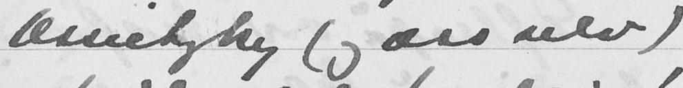Ossietzky (og oss selv),
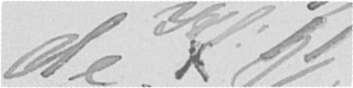de Kl: 11
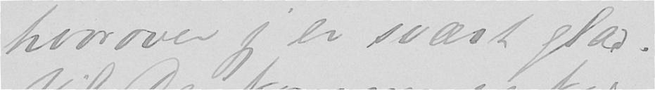hvorover jeg er svært glad!
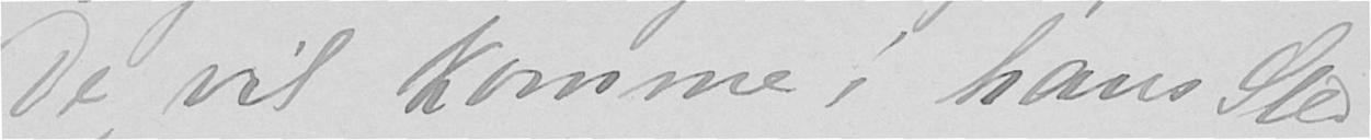De vil komme i hans Sted,
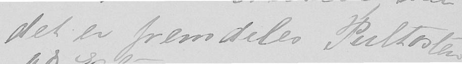det er fremdeles Pultosten
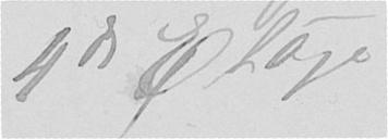4d Etage
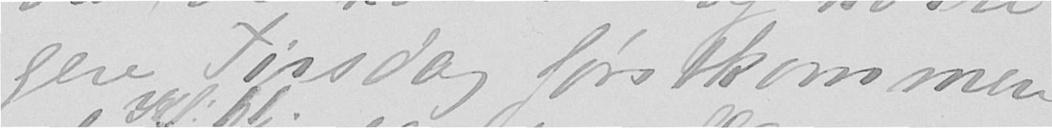gere Tirsdag førstkommen-
